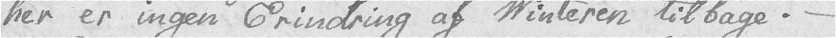her er ingen Erindring af Vinteren tilbage. –
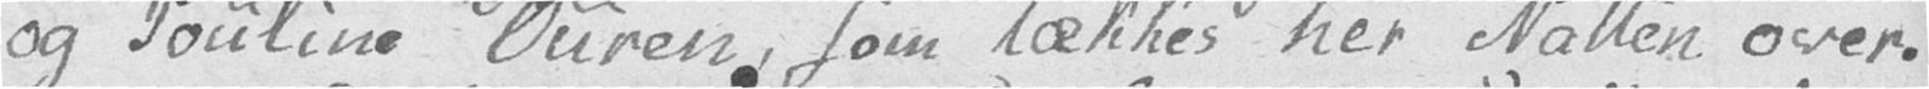og Pouline Ouren, som tækkes her Natten over.
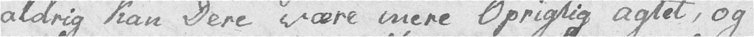aldrig kan Dere være mere Oprigtig agtet, og
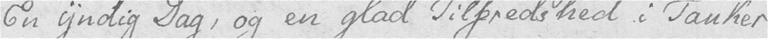En yndig Dag, og en glad Tilfredshed i Tanker
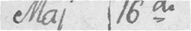Maj 16de
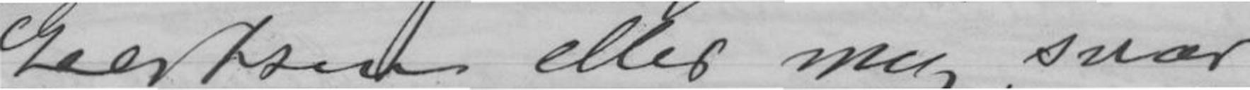Giertsen eller mig svar
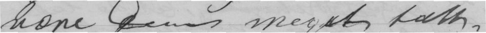være Dem meget tak-
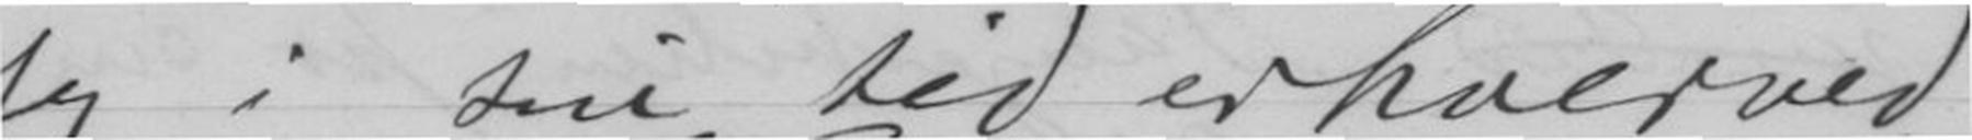jeg i sin tid erhverved
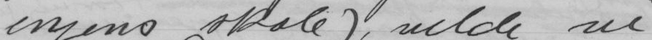engens skole), vilde vi
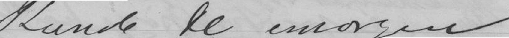Kunde de imorgen
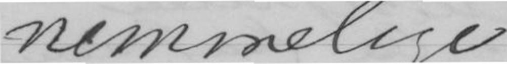nemmelige
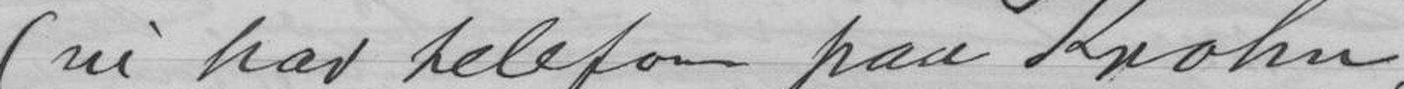(vi har telefon paa Krohn-
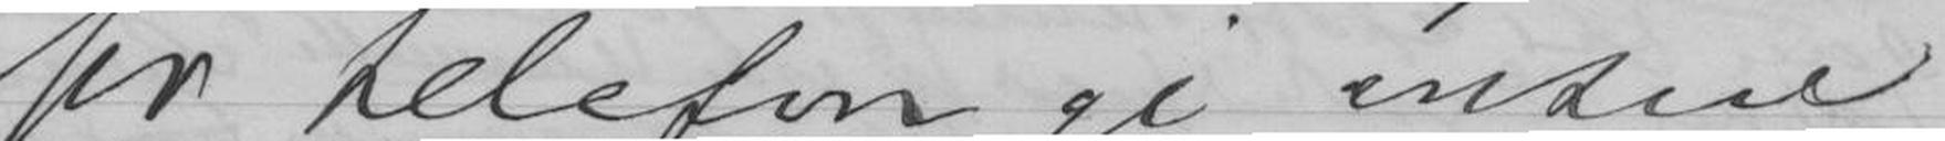pr telefon gi enten
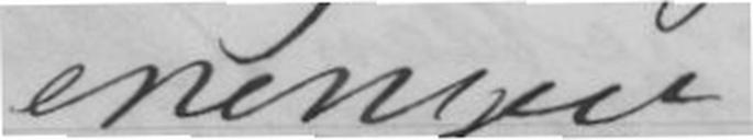eningen.
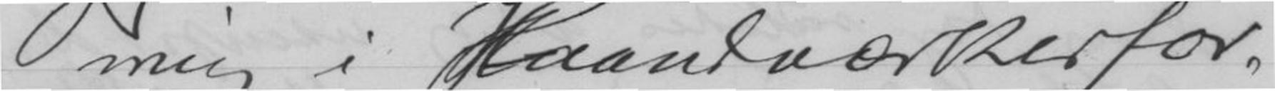mig i Haandværkerfor-
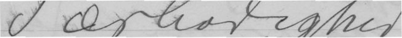I ærbødighed
